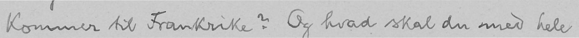kommer til Frankrike? Og hvad skal du med hele
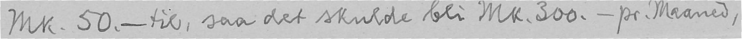Mk. 50. - til, saa det skulde bli Mk. 300. - pr. Maaned,
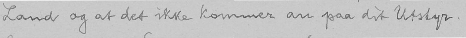Land og at det ikke kommer an paa dit Utstyr.
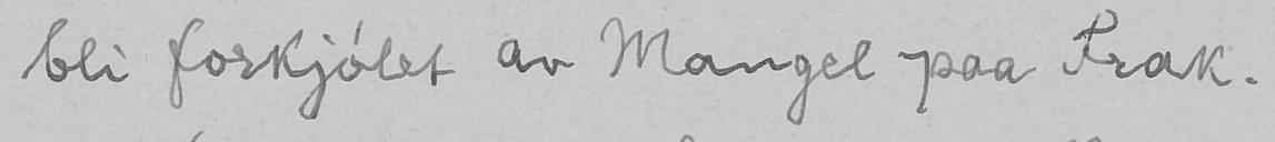bli forkjølet av Mangel paa Frak.
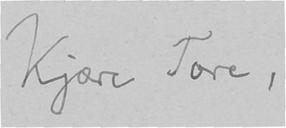Kjære Tore,
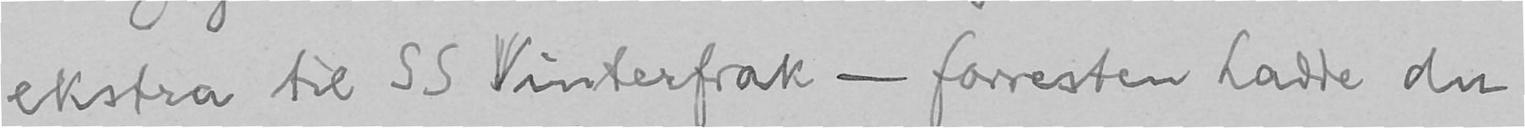ekstra til SS Vinterfrak - forresten hadde du
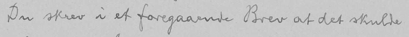Du skrev i et foregaaende Brev at det skulde
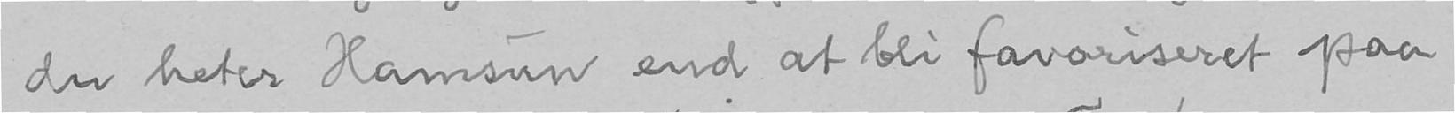du heter Hamsun end at bli favoriseret paa
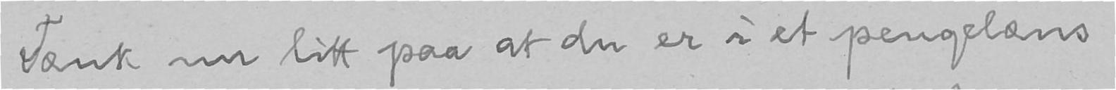Tænk nu litt paa at du er i et pengelæns
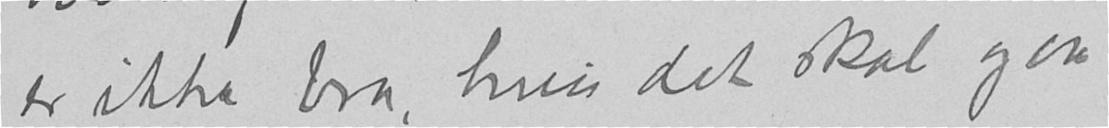er ikke bra, hvis det skal gaa
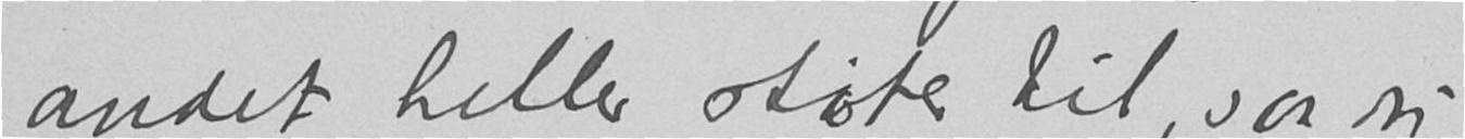andet heller støter til, saa du
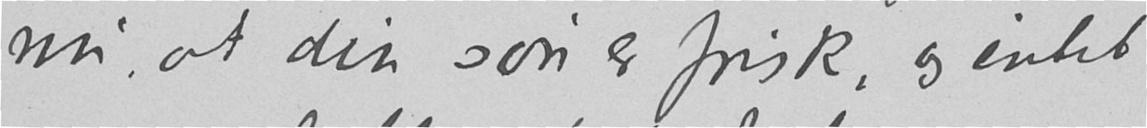nu, at din søn er frisk, og intet
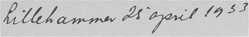Lillehammer 25 april 1933
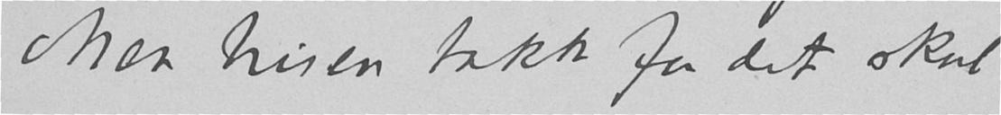Men tusen takk for det skal
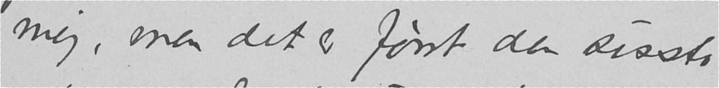mig, men det er først den sisste
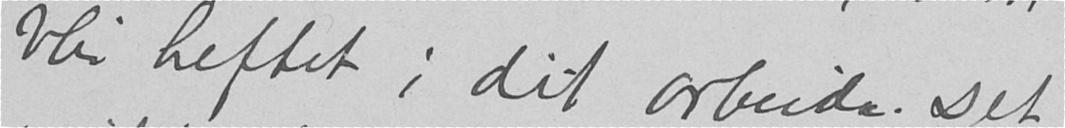blir heftet i dit arbeide. Det
